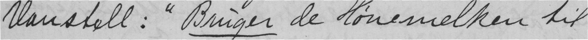Vanstell: "Bruger de Hønemelken til
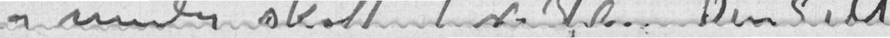og mulig skaffe fixe Ideer – Din E M
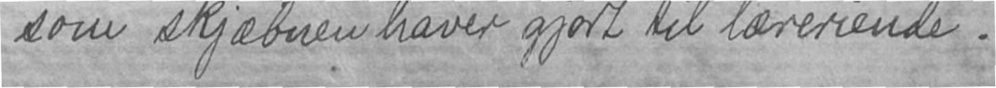som skjæbnen haver gjørt til læreriende.
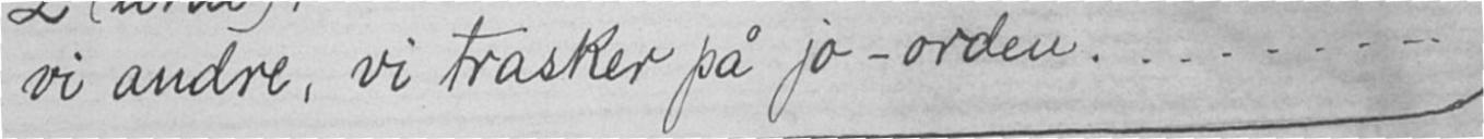vi andre, vi trasker pa jo-orden........
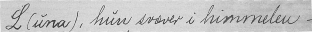L(una), hun svæver i himmelen -
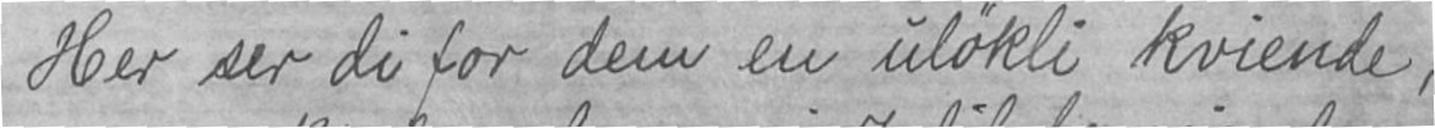Her ser di for dem en uløkli kviende,
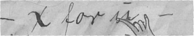- X for u -
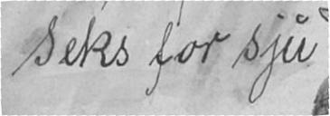Seks for sju.
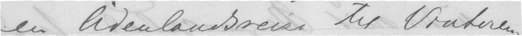en Udenlandreise til Vinteren.
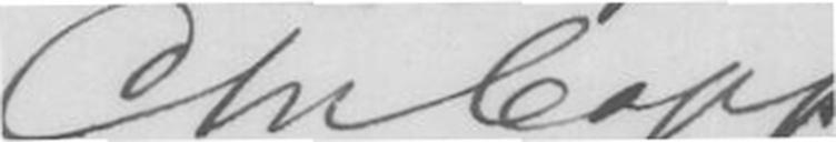Chr Cappelen
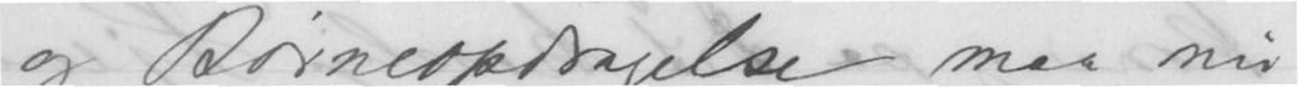og Børneopdragelse maa nu
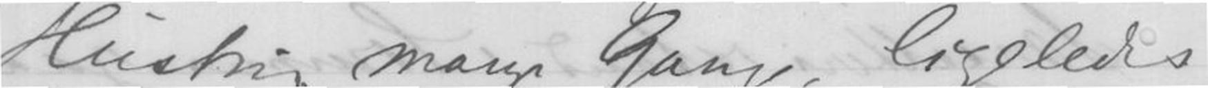Hustru mange Gange, ligeledes
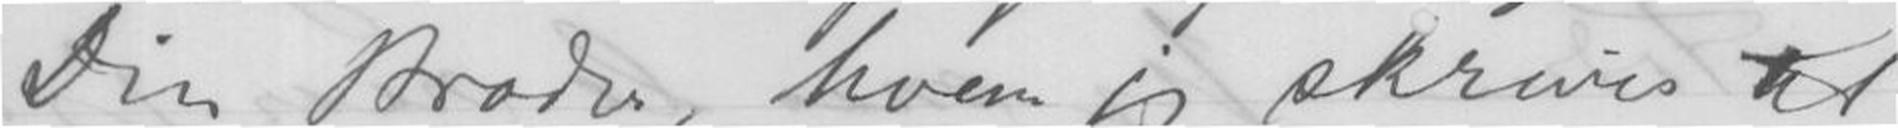Din Broder, hvem jeg skriver til
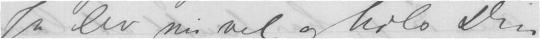Ja lev nu vel og hils Din
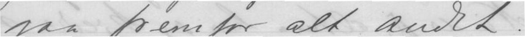gaa fremfor alt andet.
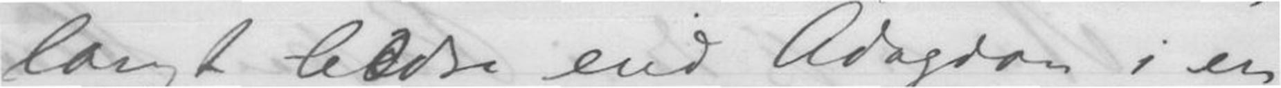langt bedre end Adogion i en
